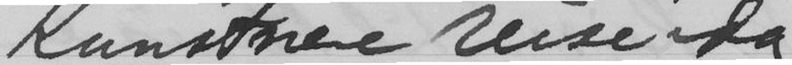kunstnere reise idag
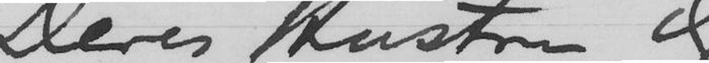Deres Hustru og
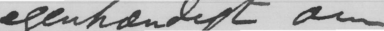egenhændigt om
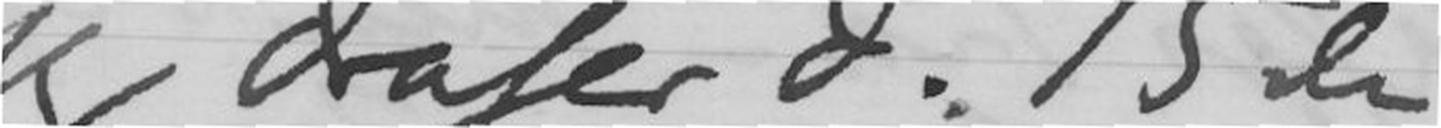- jeg drager d. 15de
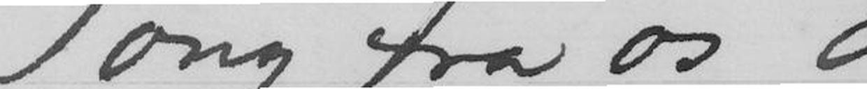Tomy fra os og
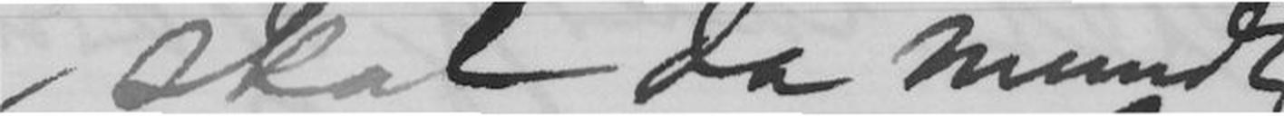og skal da mundt-
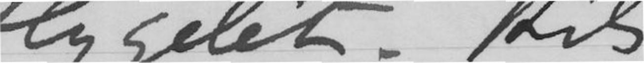Flygelet. Hils
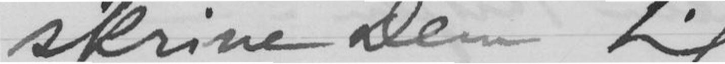skrive Dem til
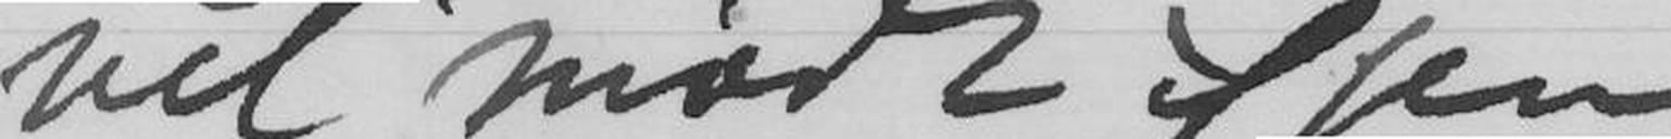vel mødt igjen
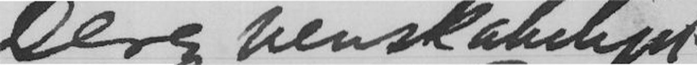Deres venskabeligst
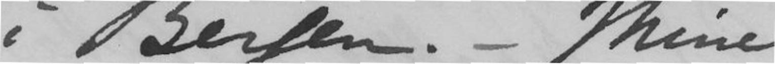i Bergen. - Mine
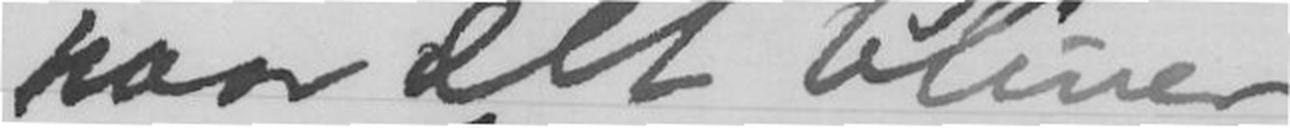naar alt bliver
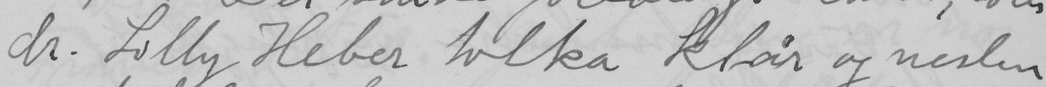dr. Lilly Heber tolka klår og nesten
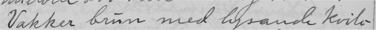Vakker brun med lysande kvite-
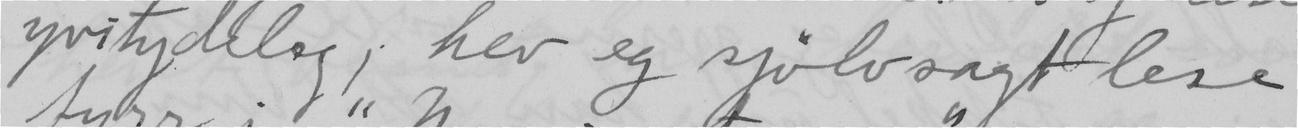yvitydeleg; hev eg sjölvsagt lese
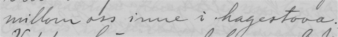millom oss inne i hagestova.
